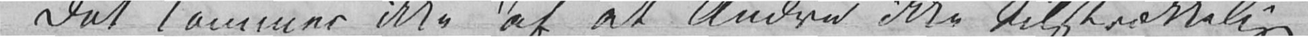Det kommer ikke af at Andre ikke tilstrækkelig
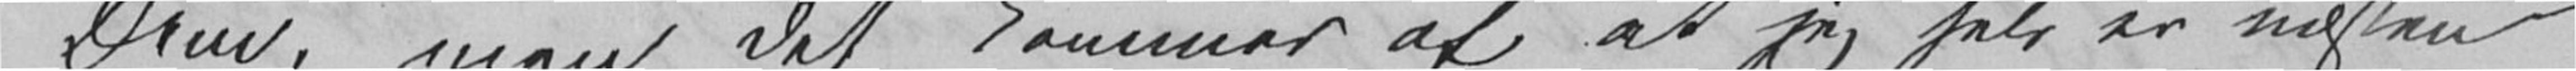Dem, men det kommer af at jeg selv er næsten
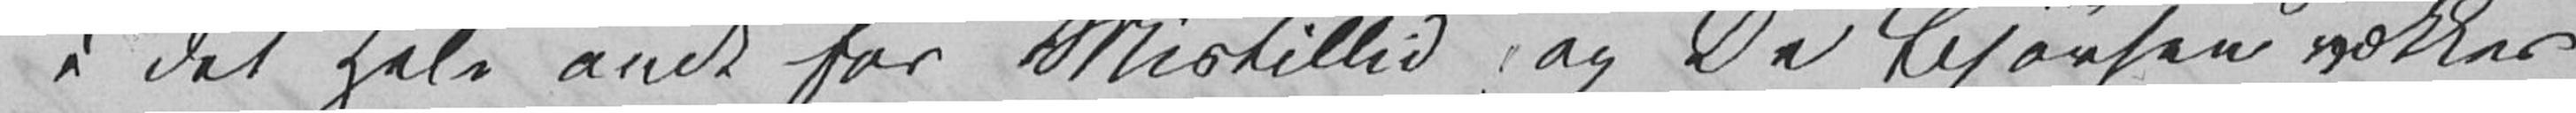i det hele ondt for Mistillid, og De Bjørnson vækker
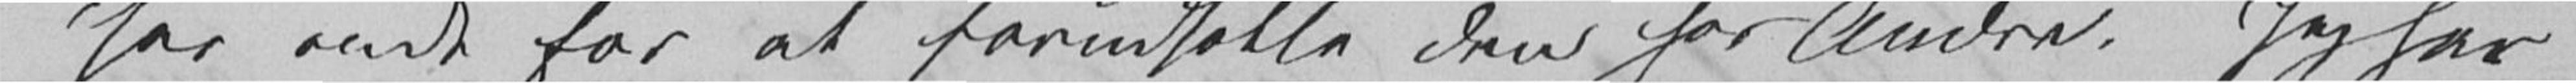har ondt for at forudsætte den for Andre. Jeg har
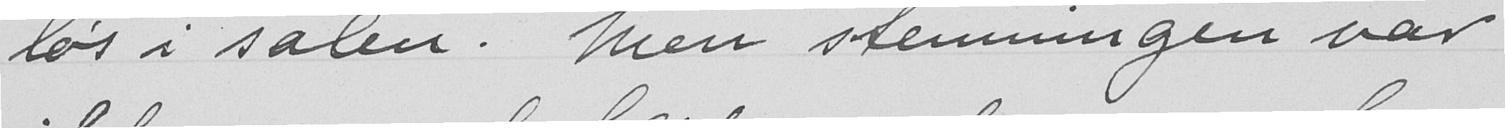løs i salen. Men stemningen var
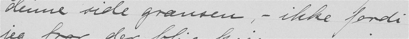denne side grænsen, - ikke fordi
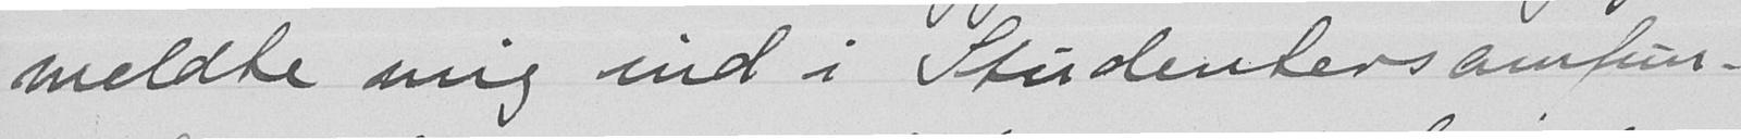meldte mig ind i Studentersamfun-
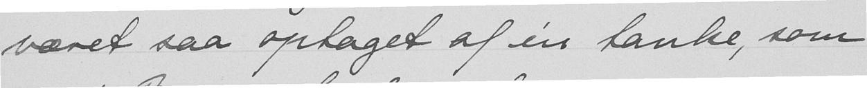været saa optaget af én tanke, som
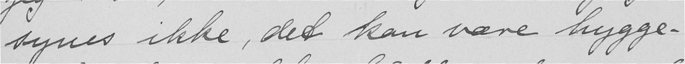synes ikke, det kan være hygge-
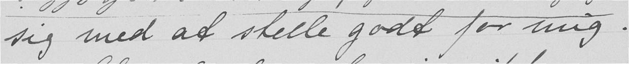sig med at stelle godt for mig.
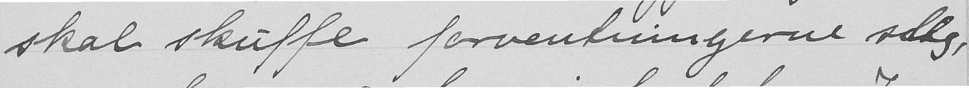skal skuffe forventningerne stadig,
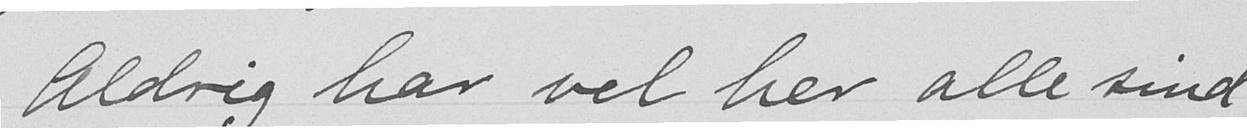Aldrig har vel her alle sind
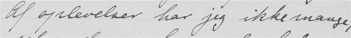Af oplevelser har jeg ikke mange,
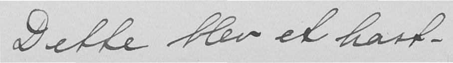Dette blev et hast-
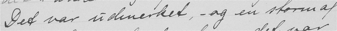Det var udmerket, - og en storm af
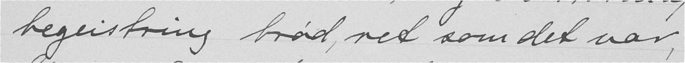begestring brød, ret som det var,
